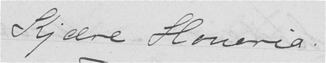Kjære Honoria!
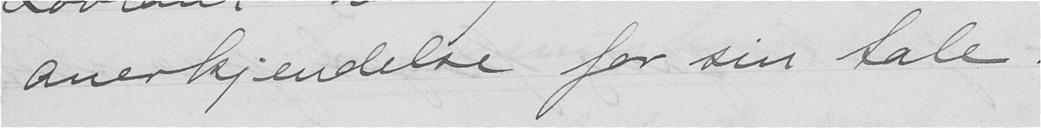anerkjendelse for sin tale.
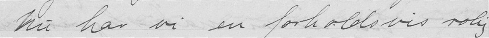Nu har vi en forholdsvis rolig
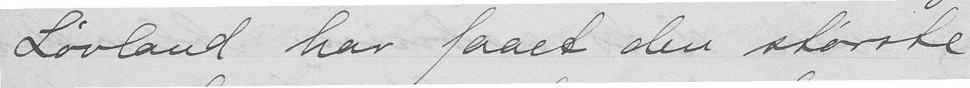Løvland har faaet den største
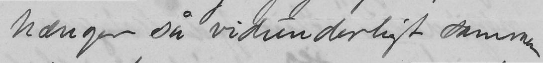hænger så vidunderligt sammen
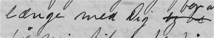længe med Dig og be
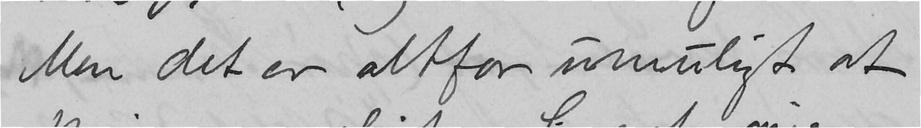Men det er altfor umuligt at
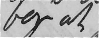for at
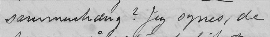sammenhæng? Jeg synes, de
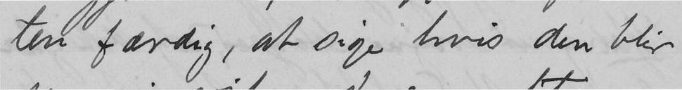ten færdig, at sige hvis den blir
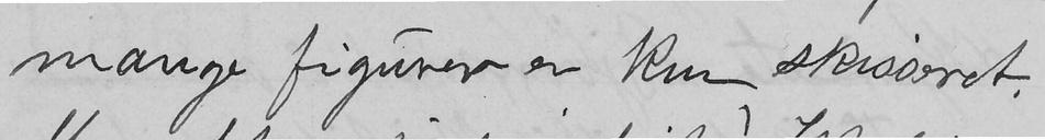mange figurer er kun skiseret.
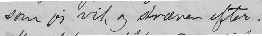som jeg vil, og stræver efter.
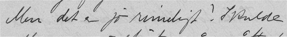Men det er jo rimeligt! Skulde
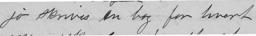jo skrives en bog for hvert
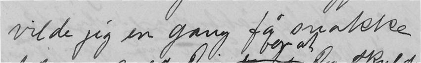vilde jeg en gang få snakke
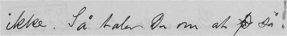ikke. Så taler Du om at f så
